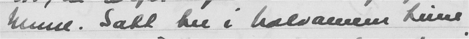henne. Satt her i halvannen time
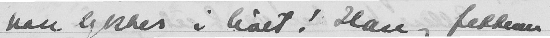han lykkes i livet! Han og fetteren
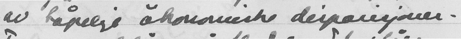av tåpelige økonomiske disposisjoner.
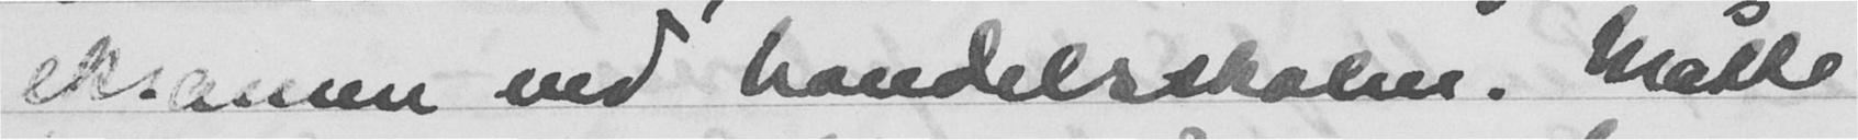eksamen ved handelsskolen. Måtte
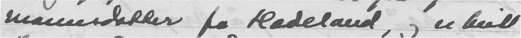mannsdatter fra Hadeland, og er blitt
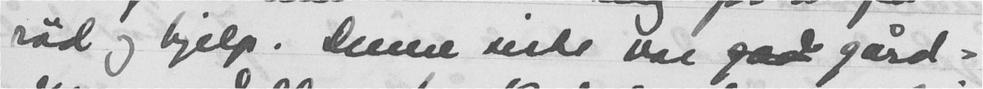nød og hjelp. Denne siste var  gård-
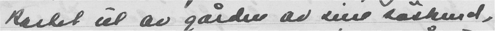kastet ut av gården av sine søskend,
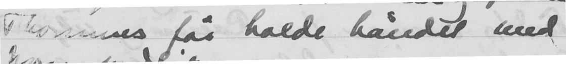Thommes får holde båndet med
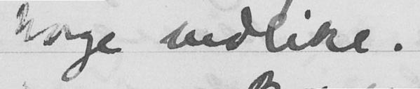Norge vedlike.
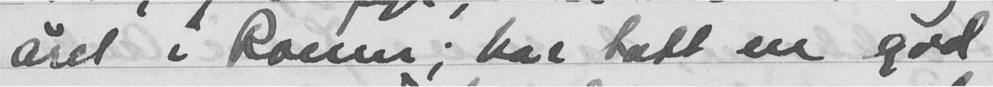året i Ronen; har tatt en god
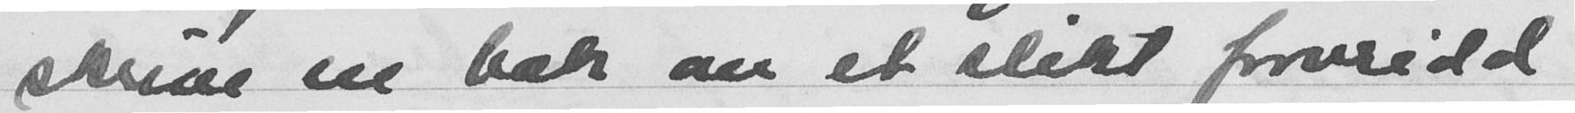skrive en bok om et slikt forvridd
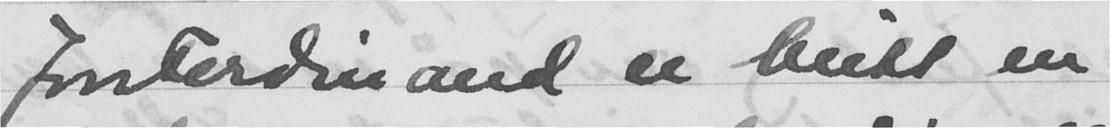FonFerdinand er blitt en
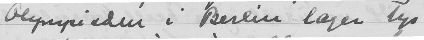Olympiaden i Berlin lager lys
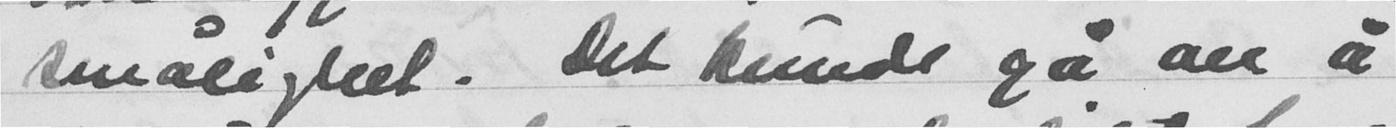småligheet. Det kunde gå an å
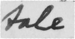tale
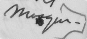Morgen -
